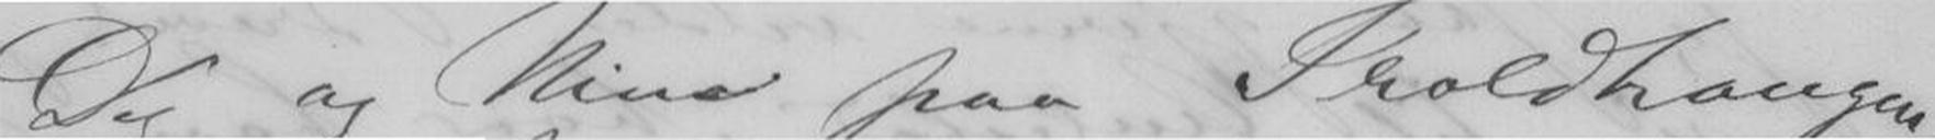Dig og Nina paa Troldhaugen,
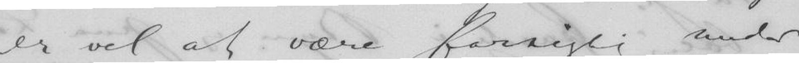er vel at være forsigtig under
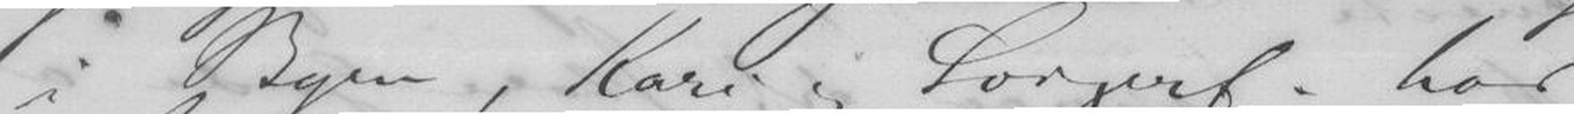i Byen, Kari og Svigerf. har
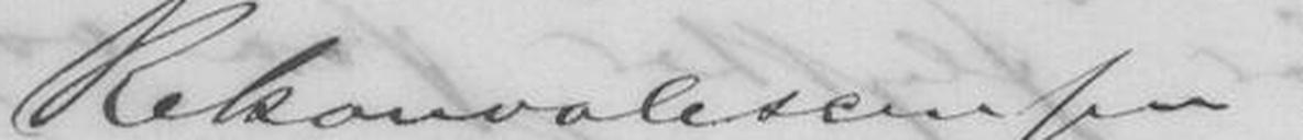Rekonvalescensen
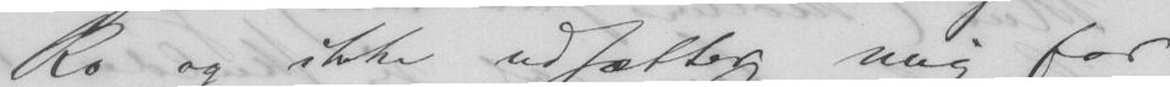Ro og ikke udsætter mig for
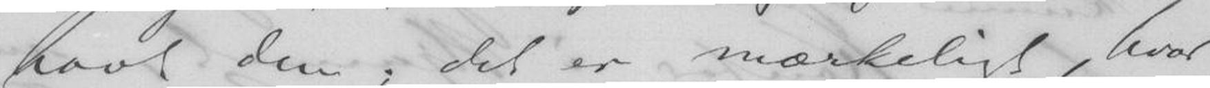havt den; det er mærkeligt, hvor
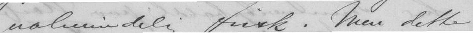ualmindelig frisk. Men dette
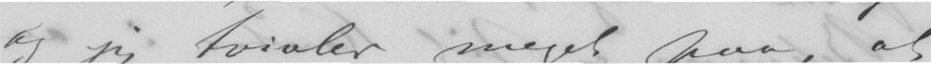og jeg tvivler meget paa, at
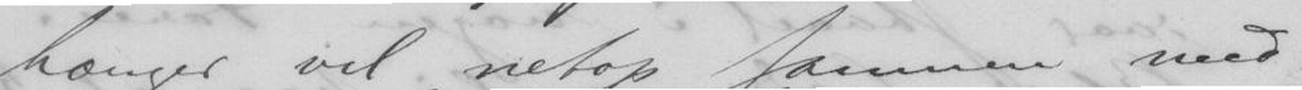hænger vel netop sammen med
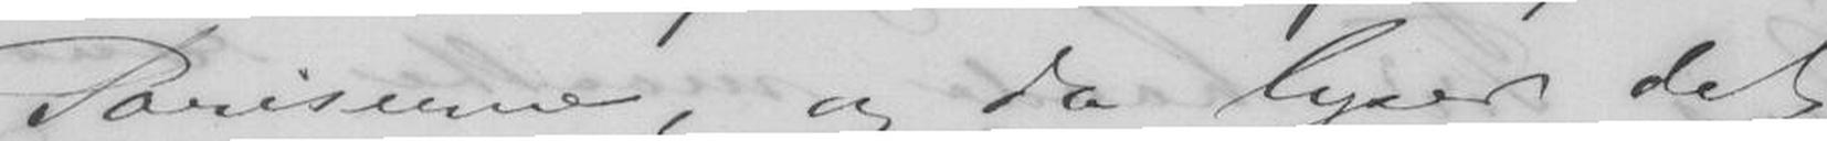Pariserne, og da lyser det
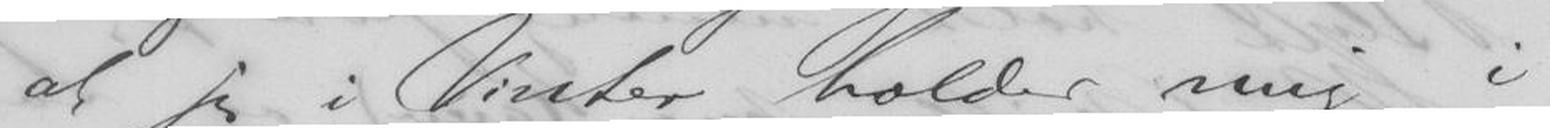at jeg i Vinter holder mig i
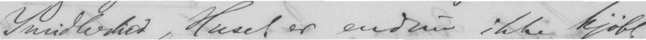Imidlertid, Huset er endnu ikke kjøbt,
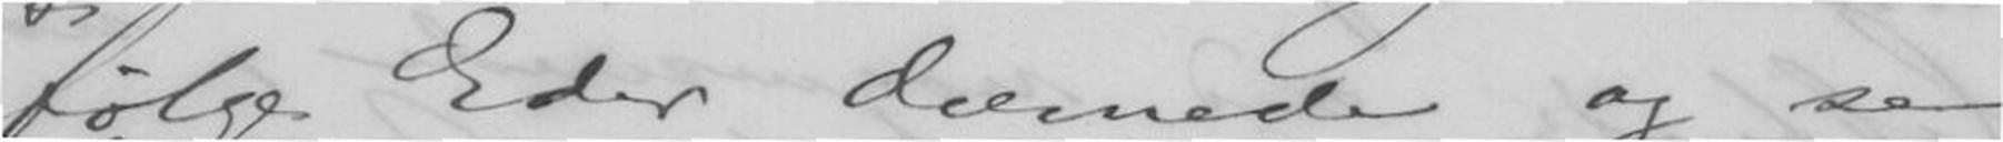følge Eder dernede og se
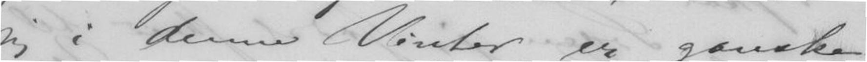jeg i denne Vinter er ganske
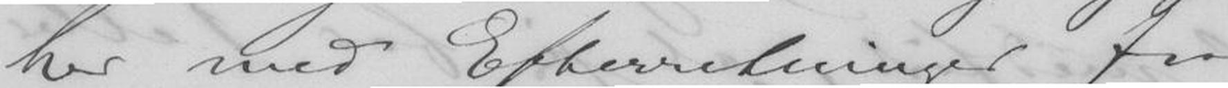her med Efterretninger fra
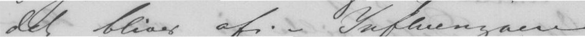det bliver af. - Influenzaen
